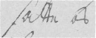satte os
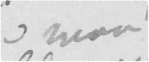saa
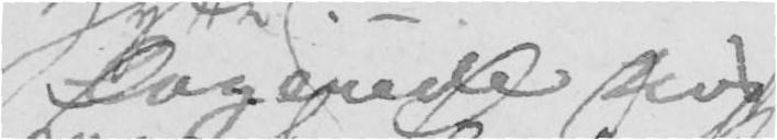liggende ved
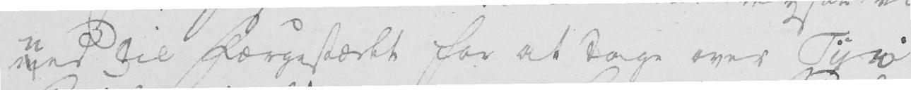ned til Færgestædet for at tage over Tyri-
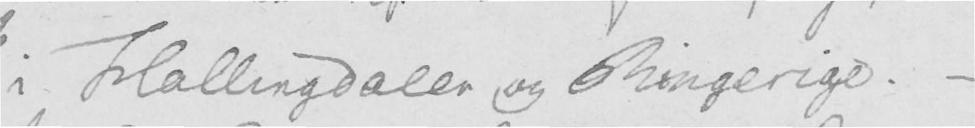i Hallingdalen og Ringerige. -
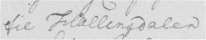til Hallingdalen
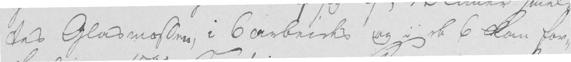tes Glasmassen, i 6 arbeides og i de 6 kan for-
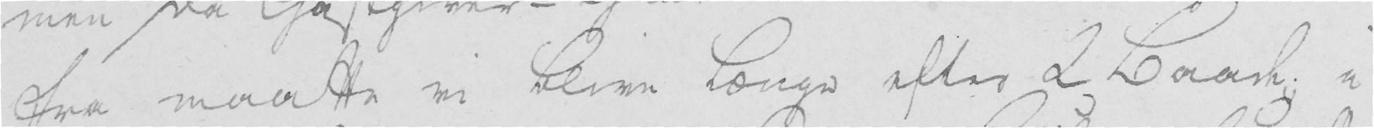fra maatte vi blive længe efter 2 Baade; i
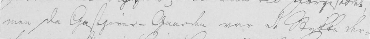men da Gastgiver-Gaarden var et Stykke der-
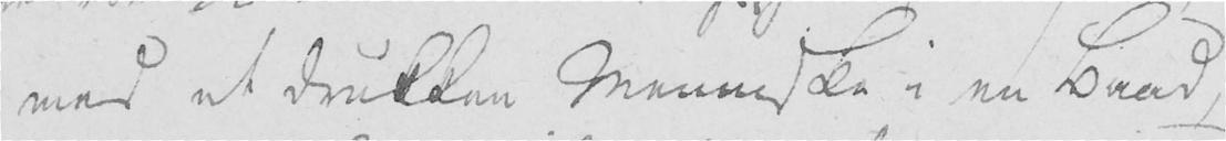med et drukken Menniske i en Baad,
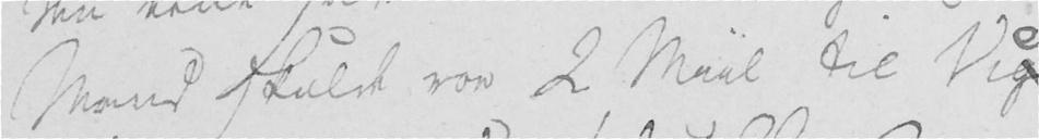Mand skulde roe 2 Miil til Vig
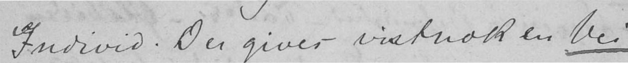Individ. Der gives vistnok en Vei-
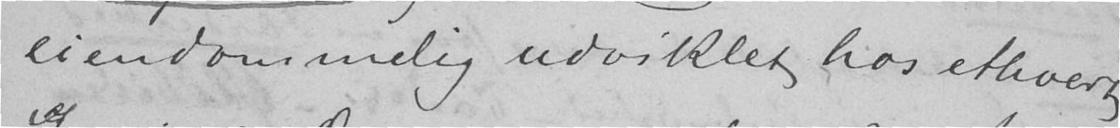eiendommelig udviklet hos ethvert
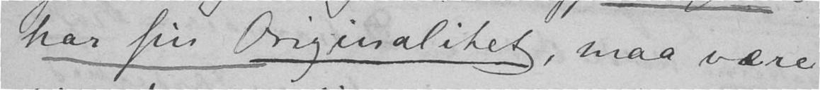har fin Originalitet, maa være
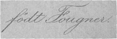født Fougner.
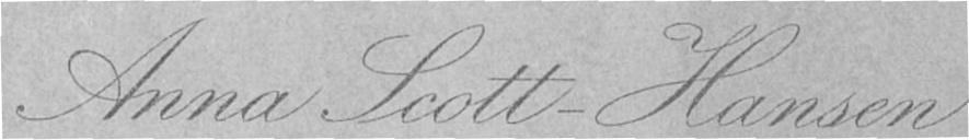Anna Scott-Hansen
